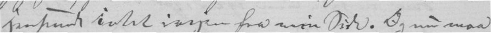henseende intet ivejen fra min Side. Og nu maa
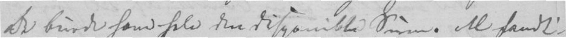De burde have hele den disponible Sum. M fandt
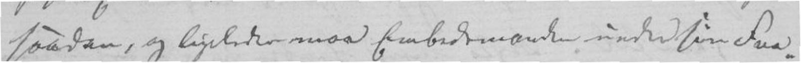saadan, og ligeledes maa Embedsmanden under sin Fra-
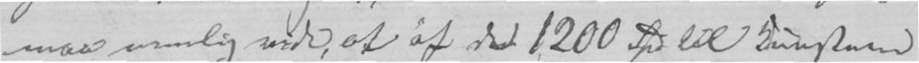maa nemlig vide, at af de 1200 Spd til Kunstneren
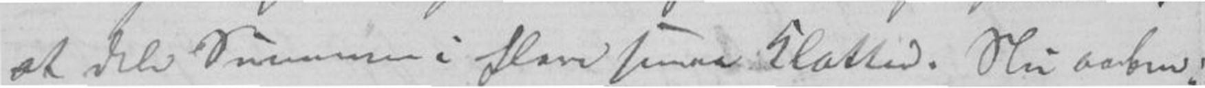at dele Summen i flere smaa Klatter. Nu aabner
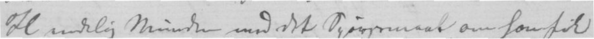H endelig Munden med det Spørgsmaal om ham fik
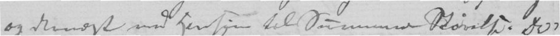og dernæst med hensyn til Summens Størrelse. De
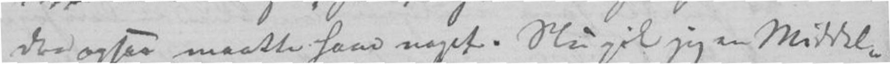andre ogsaa maatte faa noget. Nu gik jeg en Middel-
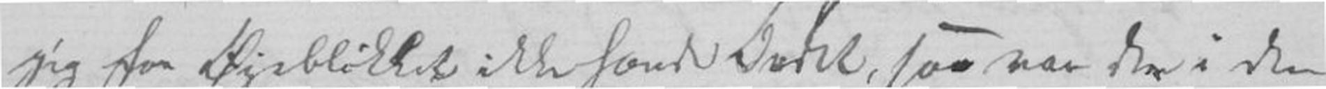jeg for Øyeblikket ikke havde Ordet, saa var der i den
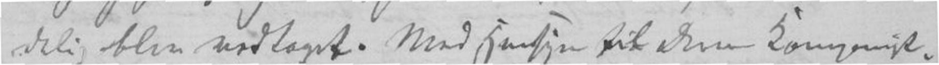delig blev vedtaget. Med hensyn til Deres Komponist
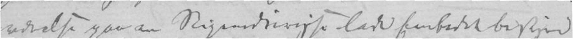tredelse gav en Stipendieeje lade Embedet bestyre
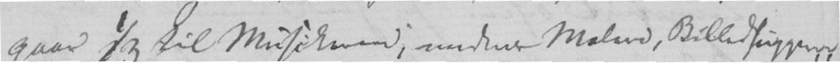gaar 1/3 til Musikere, medens Malere, Billedhuggere,
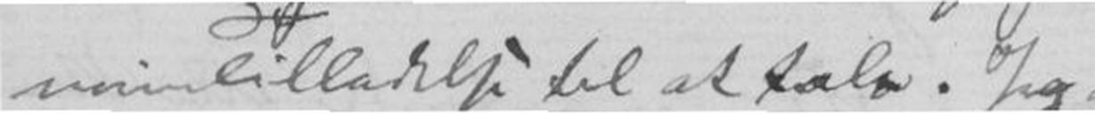min Tilladelse til at tale. Jeg
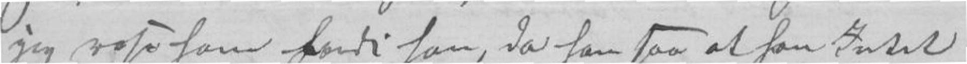jeg vise ham fordi han, da han saa at han Intet
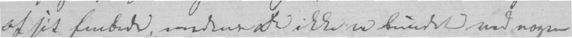af sit Embede, medens De ikke ved er bundet ved nogen
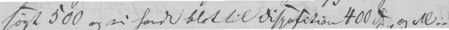søgt 500 og vi havde blot til Disposition 400 Sp, og M.
